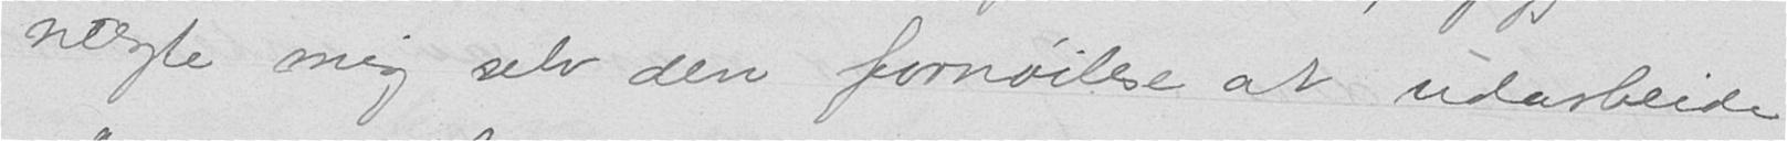nægte mig selv den fornøielse at udarbeide
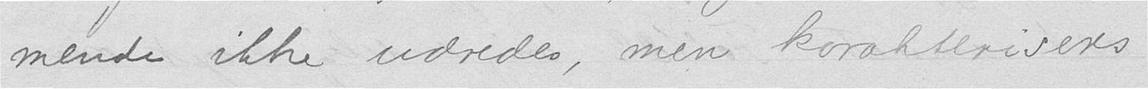mende ikke udredes, men karakteriseres
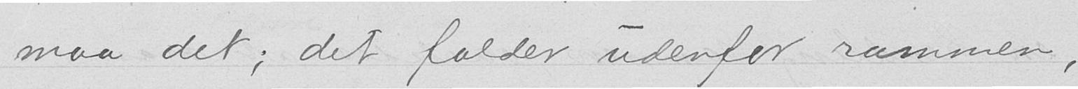maa det; det falder udenfor rammen,
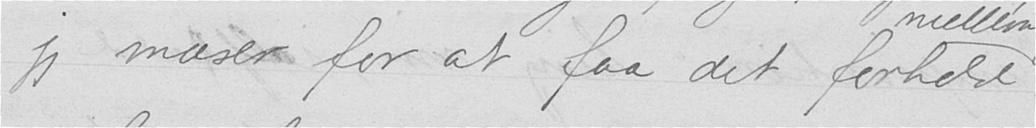jeg maser for at faa det forhold
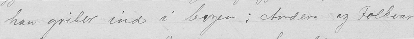han griber ind i bogen; Anders og Folkvar
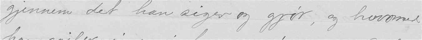gjennem det han siger og gjør, og hvormed
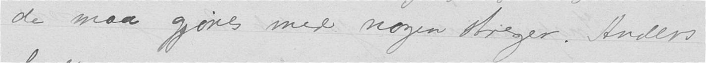de maa gjøres med nogen streger. Anders
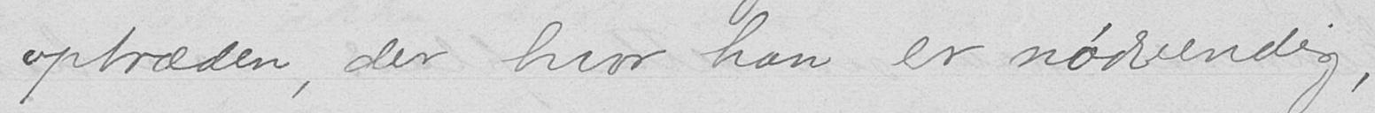optræden, der hvor han er nødvendig,
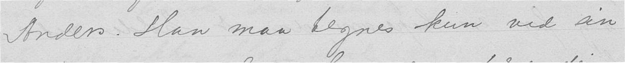Anders. Han maa tegnes kun ved sin
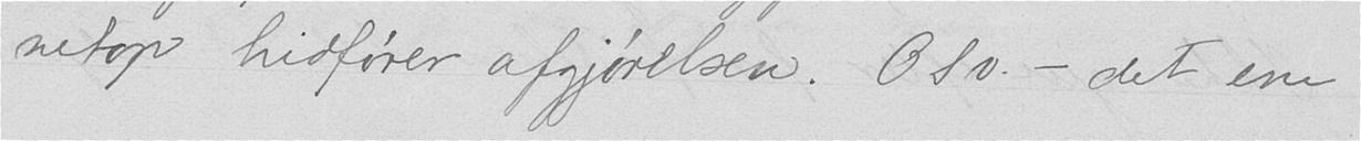netop hidfører afgjørelsen. Osv. - det ene
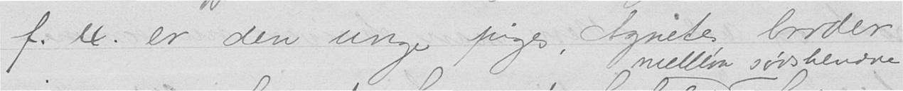f.ex. er den unge piges, Agnetes, broder,
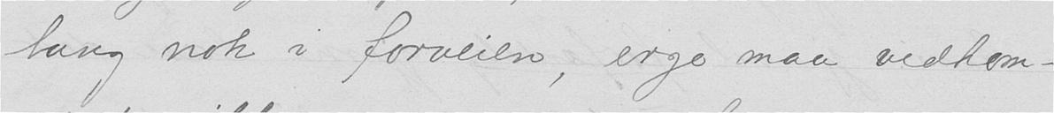lang nok i forveien, ergo maa vedkom-
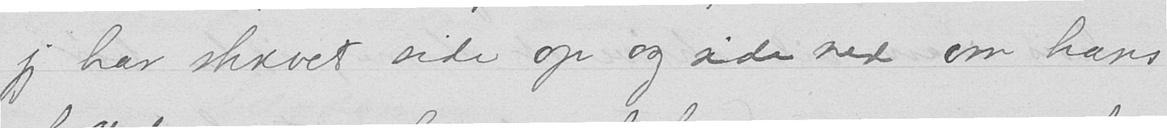jeg har skrevet side op og side ned om hans
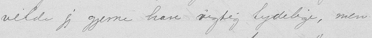vilde jeg gjerne have rigtig tydelige, men
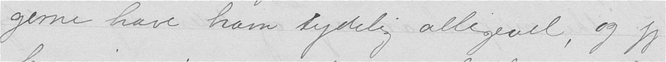gerne have ham tydelig alligevel, og jeg
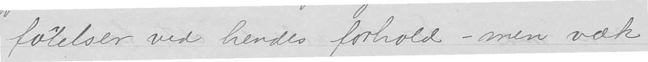følelser ved hendes forhold - men væk
